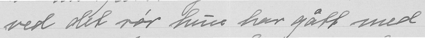ved det rør hun har gått med
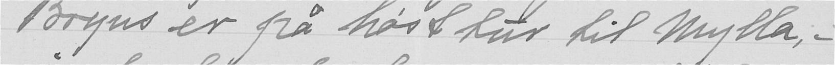Bryns er på høsttur til Mylla, -
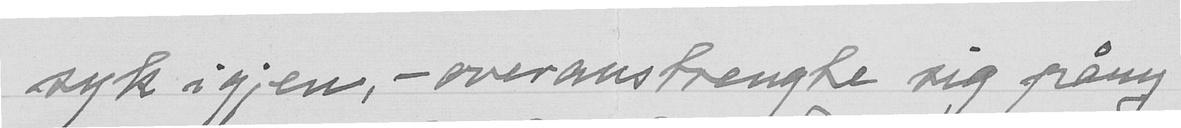syk igjen, - overanstrengte sig påny
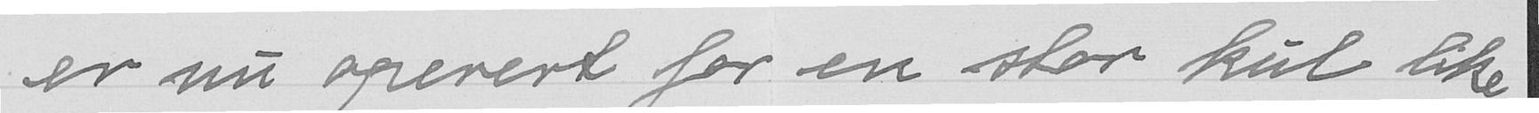er nu operert for en stor kul like
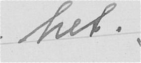het.
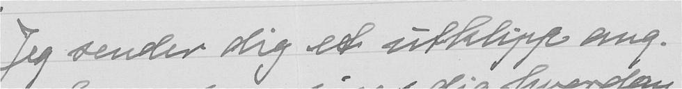Jeg sender dig et utklipp ang.
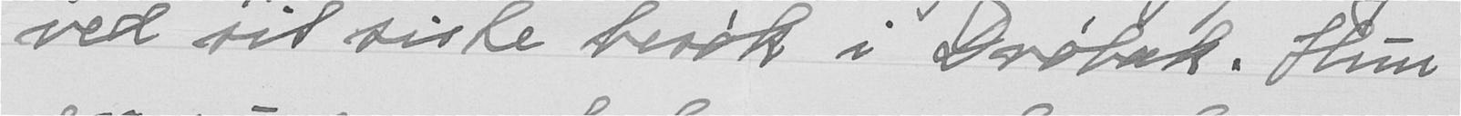ved sit siste besøk i Drøbak. Hun
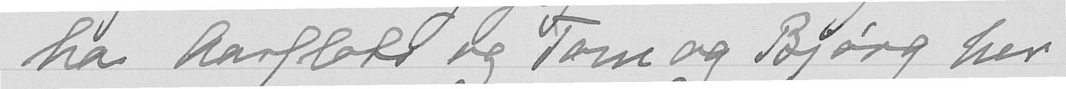ha Aarflots og Tom og Bjørg her
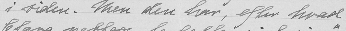i siden. Men den har, efter hvad
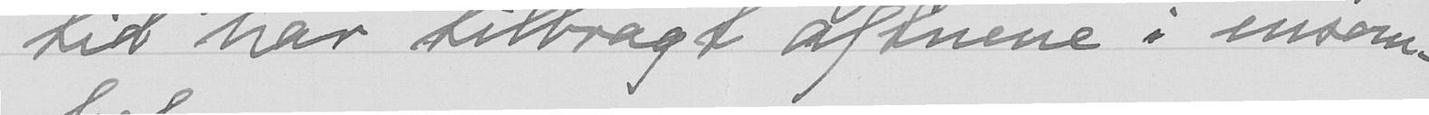tid har tilbragt aftnene i ensom-
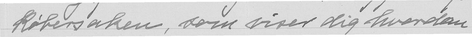Købersaken, som viser dig hvordan
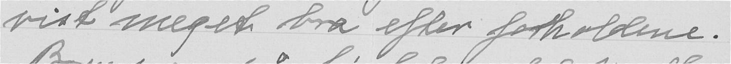vist meget bra efter forholdene.
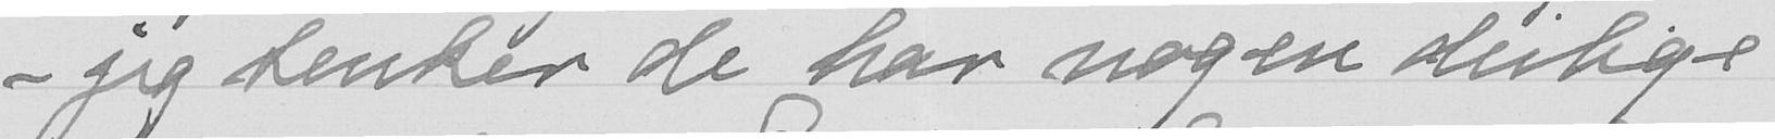- jeg tenker de har nogen deilige
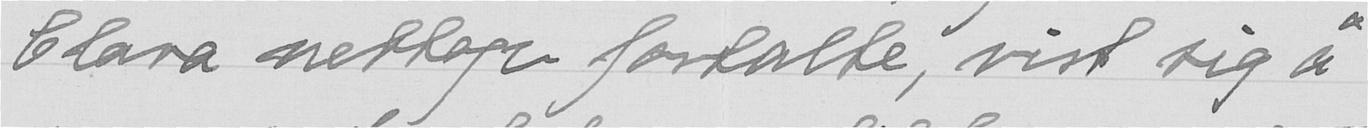Clara nettop fortalte, vist sig å
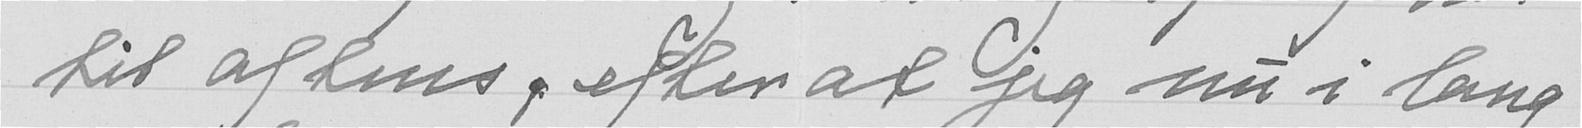til aftens, etter at jeg nu i lang
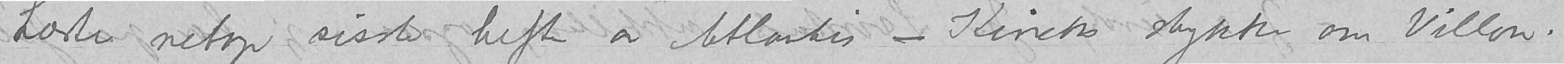Læste netop sisste hefte av Atlantis – Kincks stykke om Villon.
